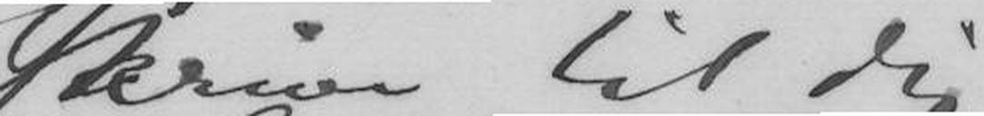jeg skrive til dig.
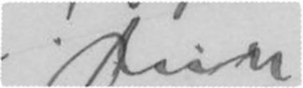Din
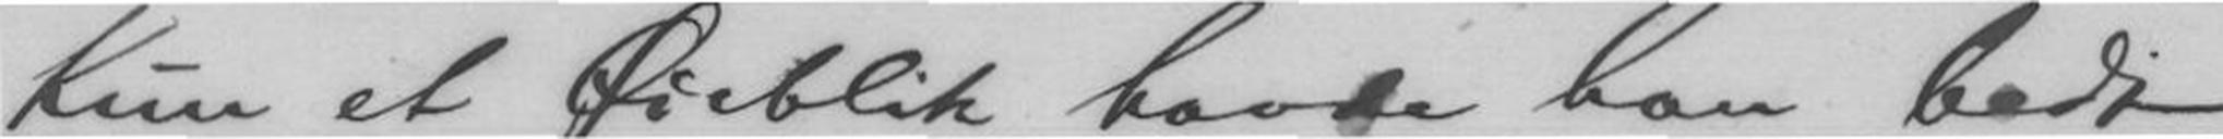Kun et Øieblik havde han bedt
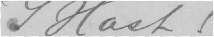I Hast!
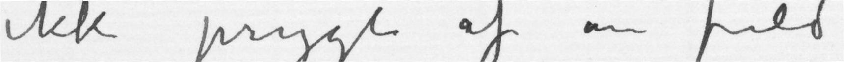ikke prygle af en fuld
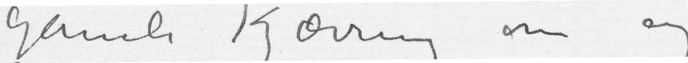gamle Kjærring om og
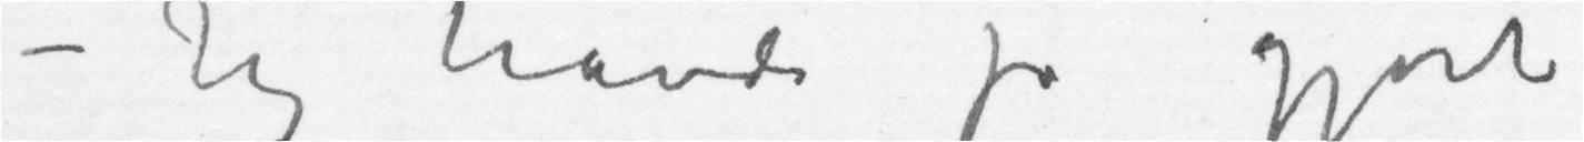– Jeg havde jo gjort
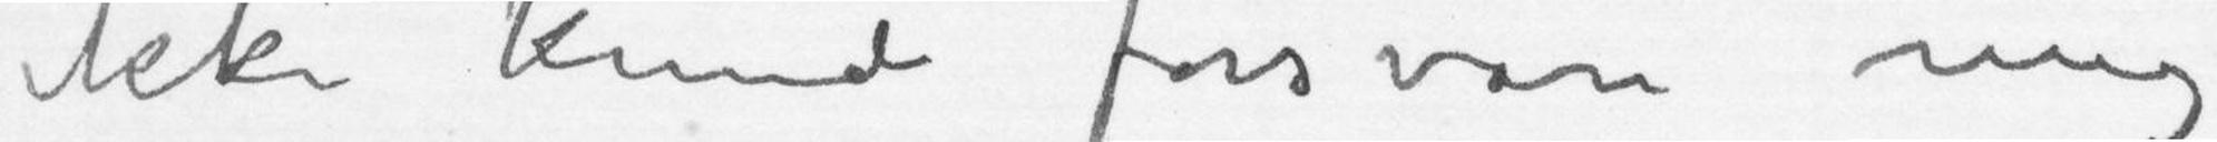ikke kunde forsvare mig
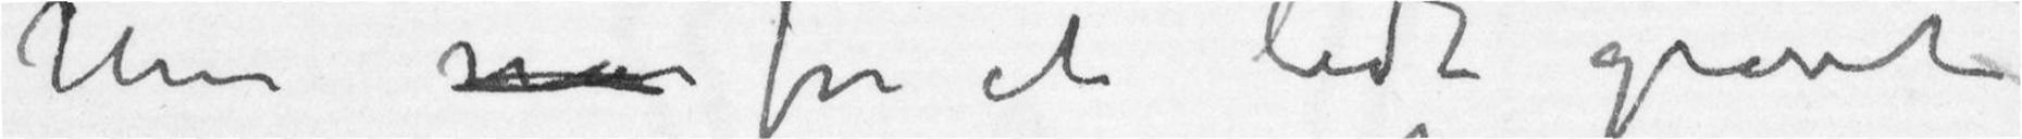Men når for et lidt grovt
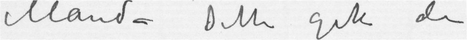Mand – Dette gik den
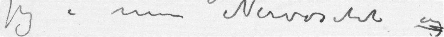jeg i min Nervøsitet og
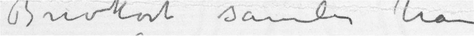Brevkort samler han
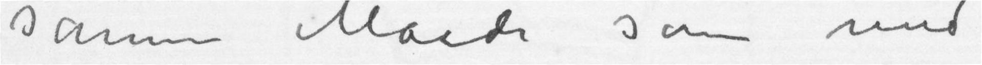samme Maade som med
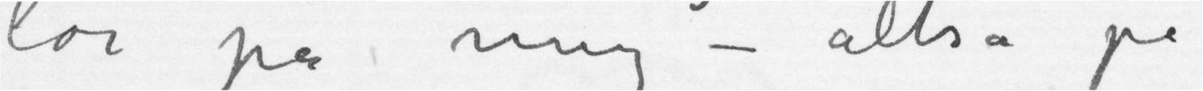løi på mig – altså på
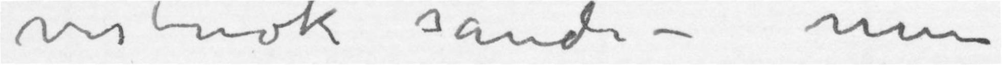vistnok sande – men
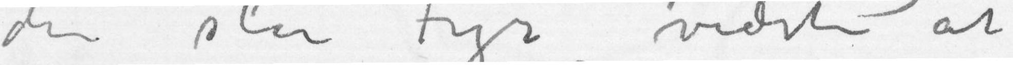den store Fyr vidste at
